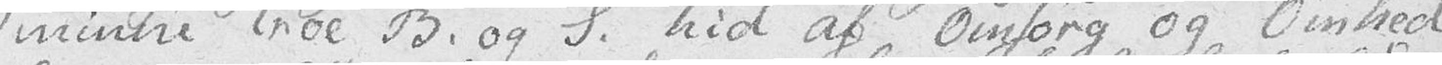minne troe B. og S. hid af Omsorg og Ømhed
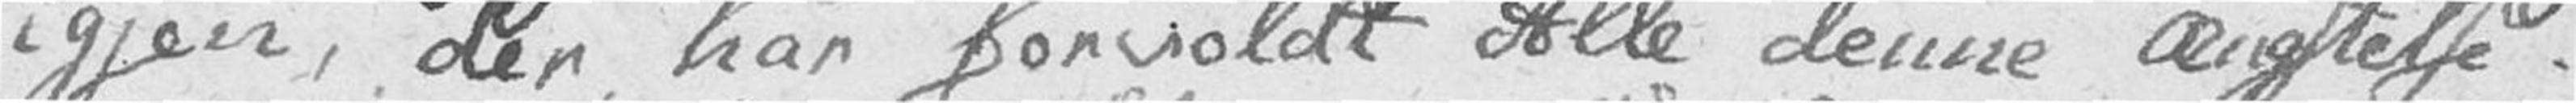igjen, der har forvoldt Alle denne ængstelse.
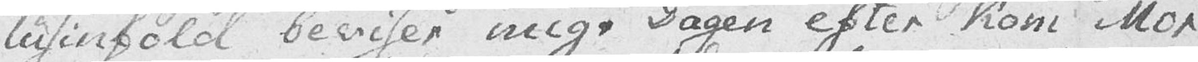tusinfold beviser mig. Dagen efter kom Mor
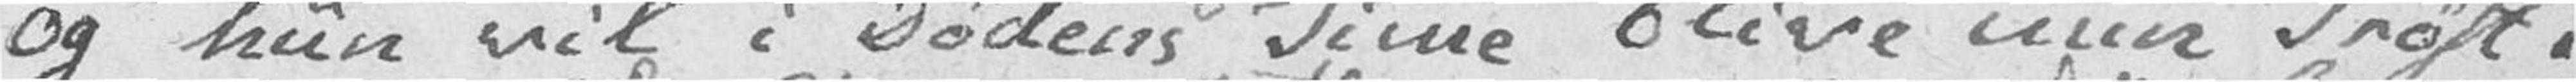og hun vil i Dødens Time blive min Trøst.
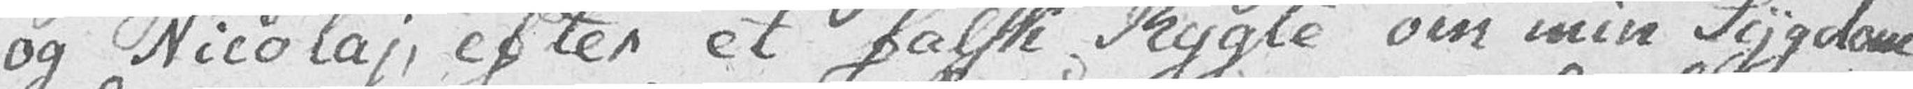og Nicolaj, efter et falsk Rygte om min Sygdom
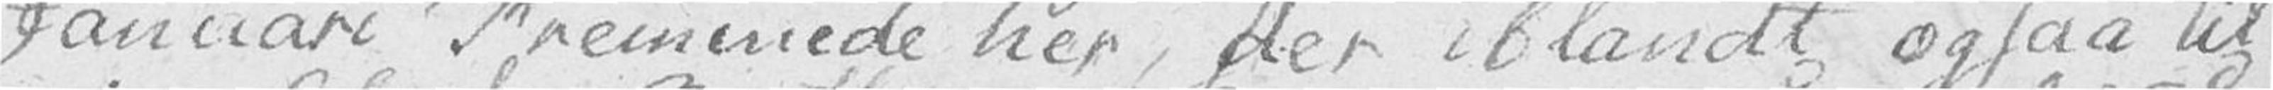Januari Fremmede her, der iblandt ogsaa til
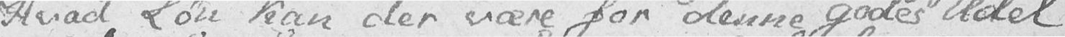Hvad Løn kan der være for denne godes Ædel
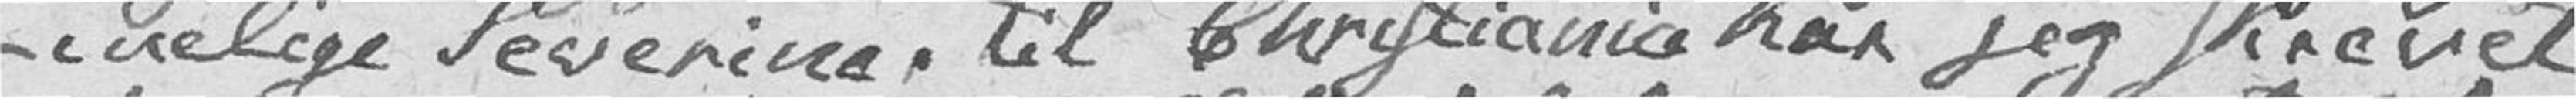-melige Severine, til Christiania har jeg skrevet
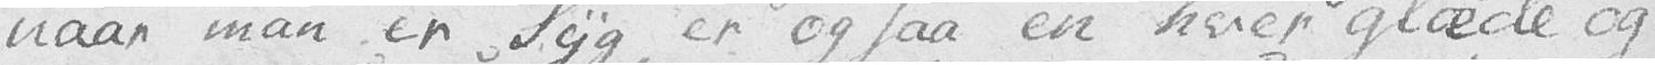naar man er Syg er ogsaa en hver glæde og
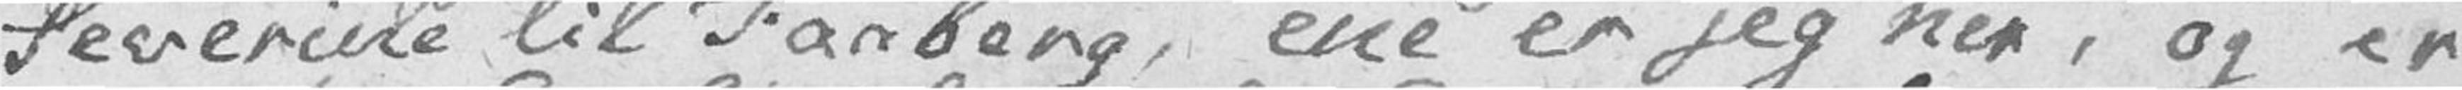Severine til Faaberg, ene er jeg her, og er
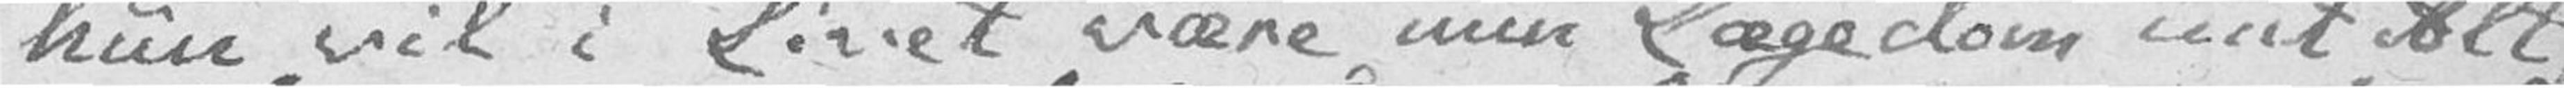hun vil i Livet være min Lægedom mit Alt,
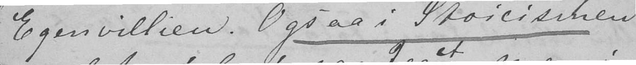Egenvillien. Ogsaa i Stoicismen
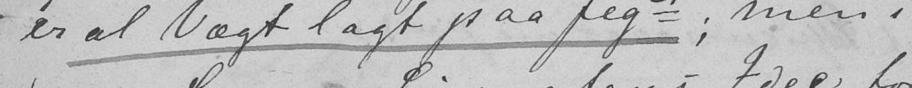er al Vægt lagt paa Jeget; men i
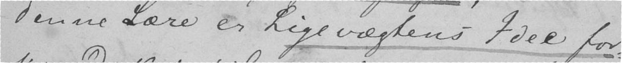denne Lære er Ligevægtens Idee for-
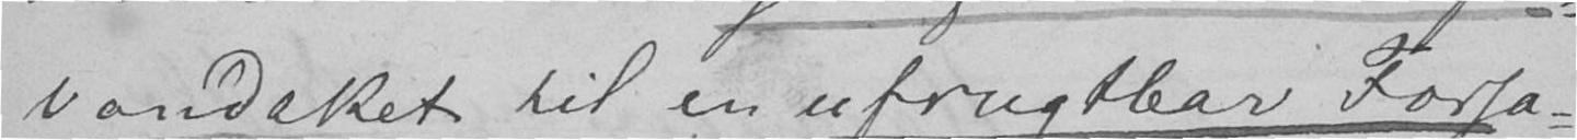vandsket til en ufrugtbar Forsa-
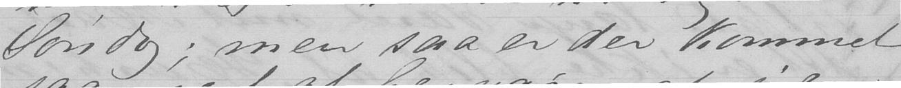Søndag; men saa er der kommet
